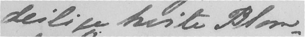deilige hvite Blom-
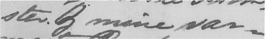ster. Og mine var-
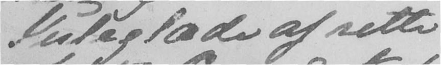Juleglæde af rette
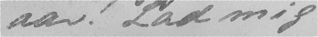aar! Lad mig
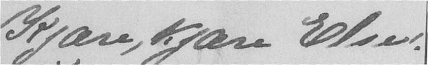Kjære, kjære Else!
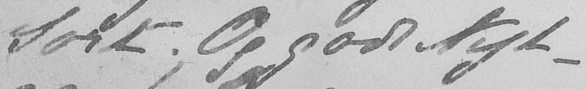Sort. Og godt Nyt-
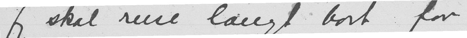Jeg skal reise langt bort for
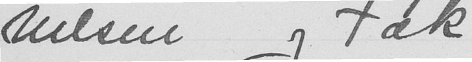hilse og tak
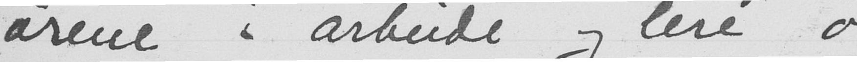ørene i arbeide og lere og
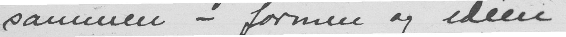sammen - formen og ideen
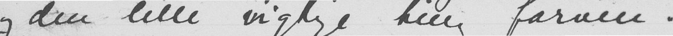og den lille vigtige ting farven.
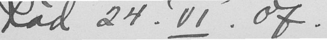Rød 24.VI.07
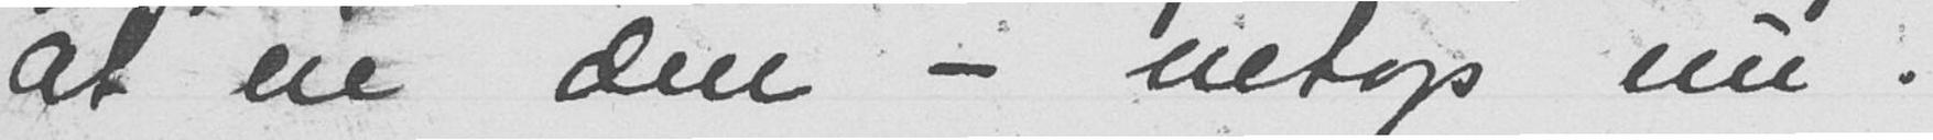at eie den - netop nu.
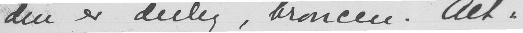den er deilig, broncen. Alt-
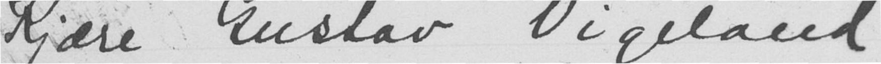Kjære Gustav Vigeland
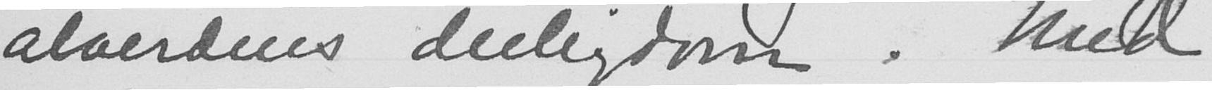alverdens deiligdom. Med
